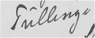Tullinge
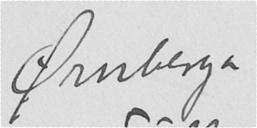Ørnberga
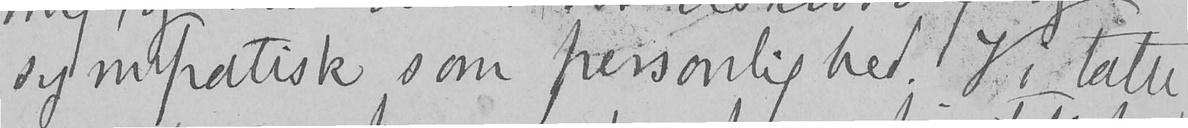sympatisk som personlighed. Vi talte
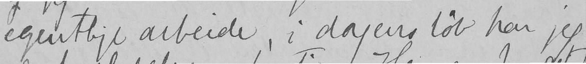egentlige arbeide, i dagens løb har jeg
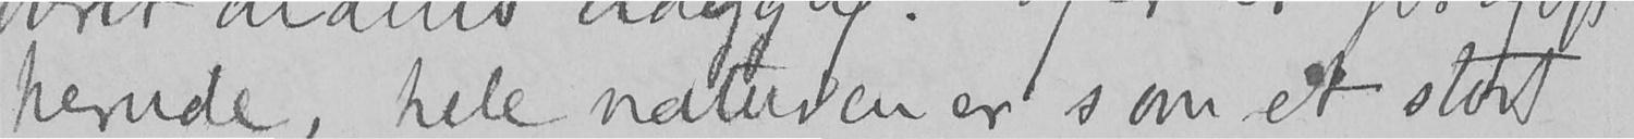herude, hele naturen er som et stort
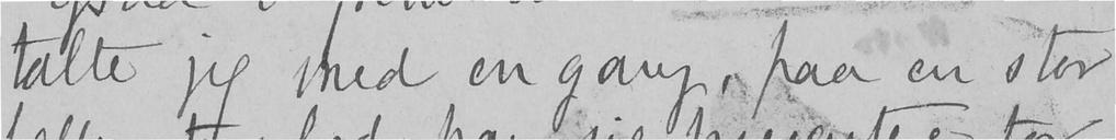talte jeg med en gang, paa en stor
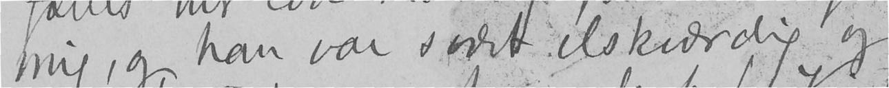mig, og han var svært elskværdig og
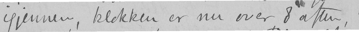igjennem, klokken er nu over 8 aften,
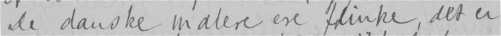De danske malere ere flinke, det er
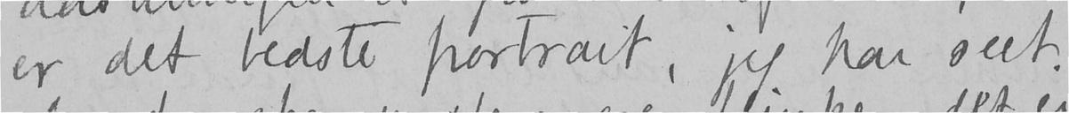er det bedste portrait, jeg har seet.
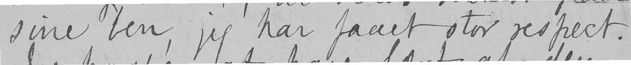sine ben, jeg har faaet stor respect.
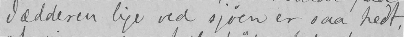Jædderen lige ved sjøen er saa hedt,
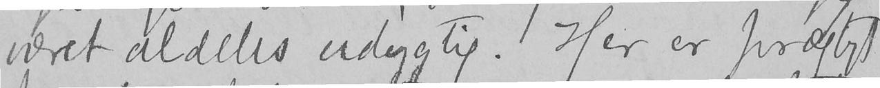været aldeles udygtig. Her er prægtigt
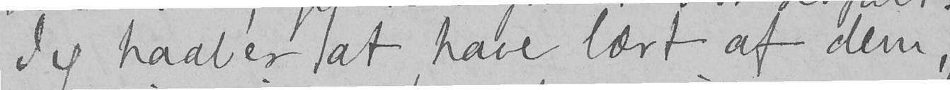Jeg haaber at have lært af dem,
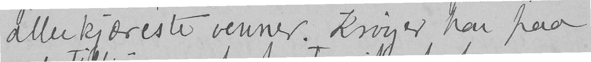aller kjæreste venner. Krøger har paa
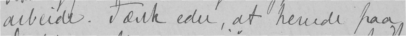arbeide. Tænk eder, at hernede paa
